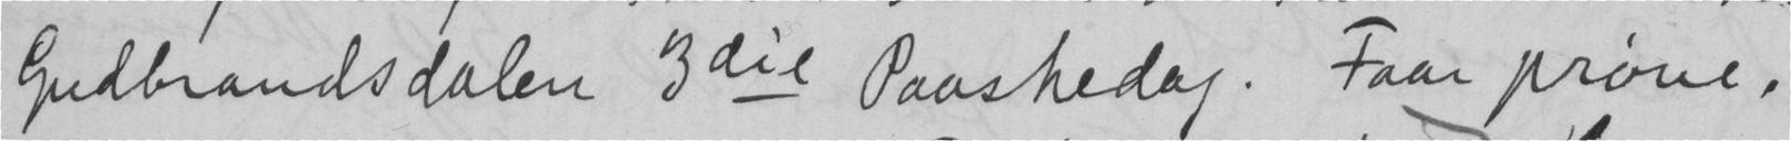Gudbrandsdalen 3die Paaskedag. Faar prøve.
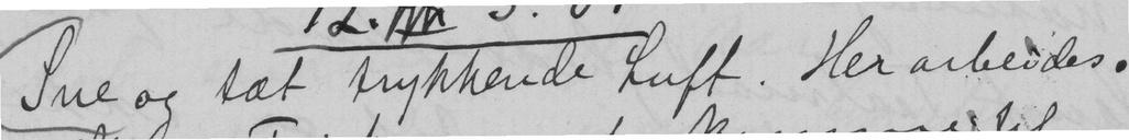Sne og tæt trykkende Luft. Her arbeides.
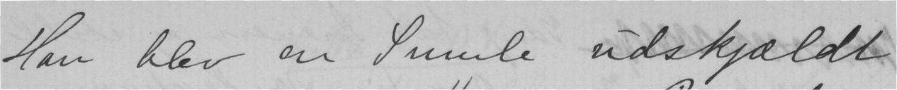Han blev en Smule udskjældt
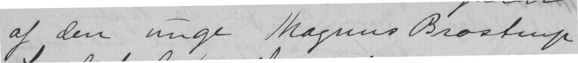af den unge Magnus Brostrup
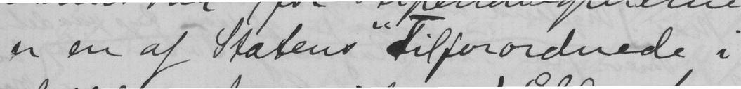er en af Statens "tilforordnede i
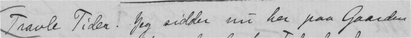Travle Tider. Jeg sidder nu her paa Gaarden
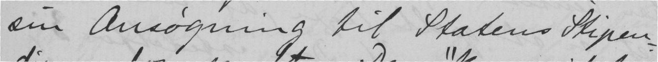sin Ansøgning til Statens Stipen-
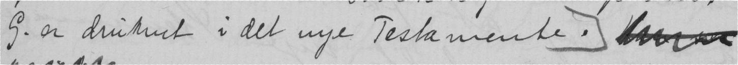G. er druknet i det nye Testamente.
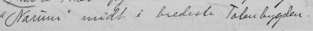"Narum" midt i bredeste Totenbygden.
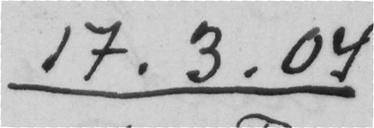17.3.04
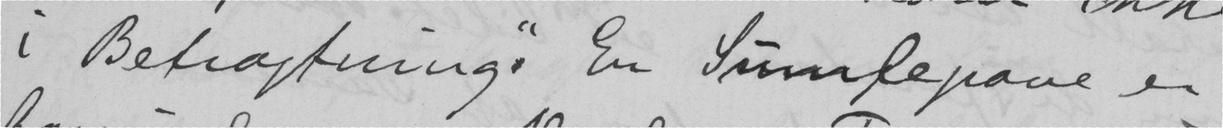i Betragtning". En Sumlepave er
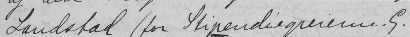Landstad (for Stipendiegreierne. G.
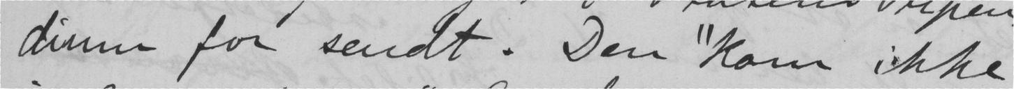dium for sendt. Den "kom ikke
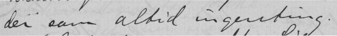der som altid ingenting.
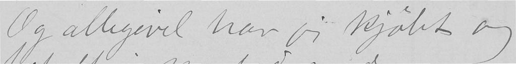Og alligevel har jeg kjøbt og
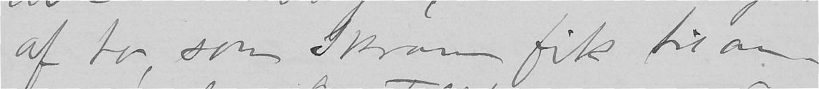af to, som Skram fik til an-
